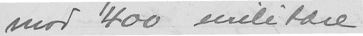mod 400 militære
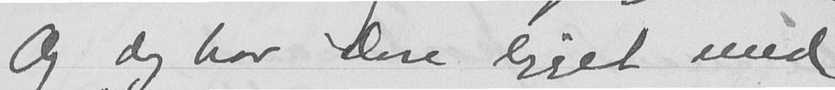Og dog har Don ligget med
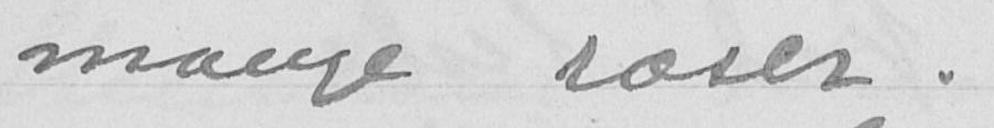mange ræser.
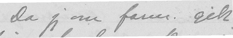Da jeg om form. gik
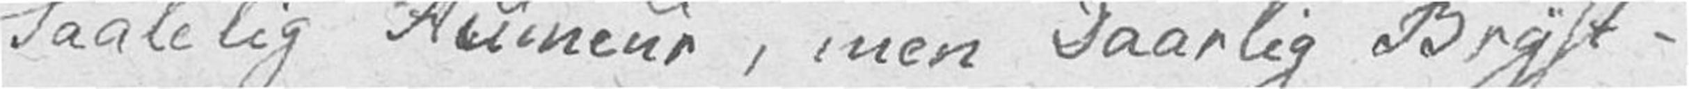Taalelig Humeur, men Daarlig Bryst.
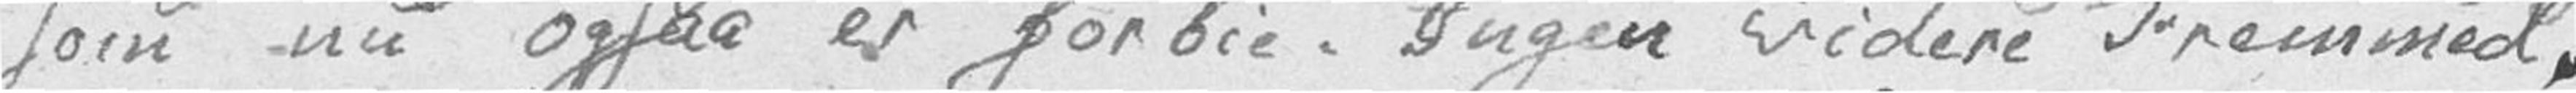som nu ogsaa er forbie. Ingen videre Fremmed,
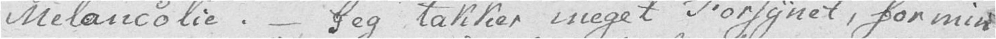Melancolie. – Jeg takker meget Forsynet, for min
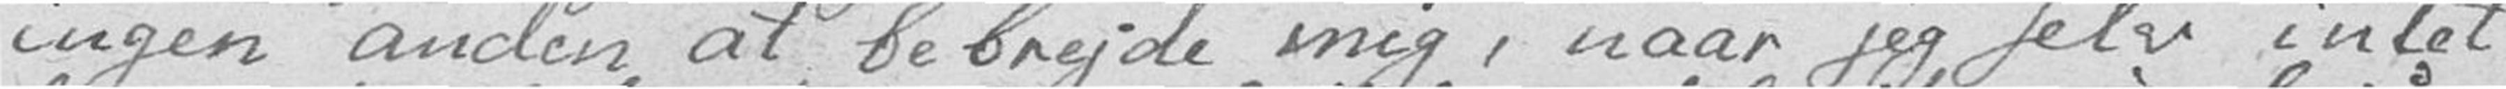ingen anden at bebrejde mig, naar jeg selv intet
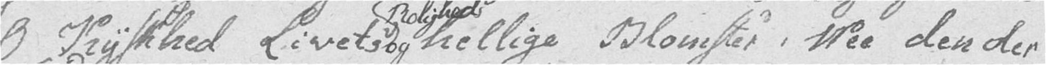O Kyskhed Livets Roligheds og hellige Blomster, Vee den der
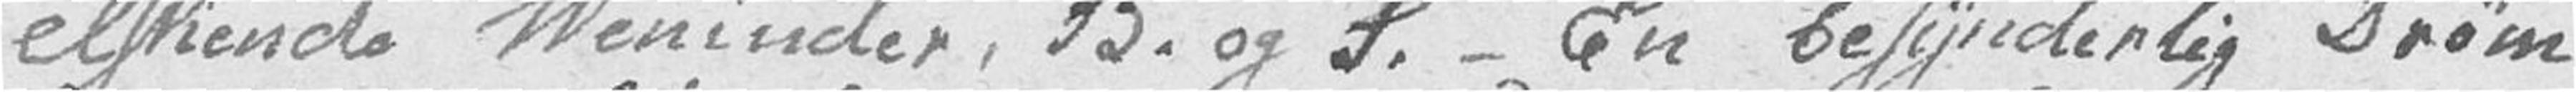elskende Veninder, B. og S. – En besynderlig Drøm
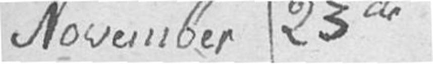November 23de
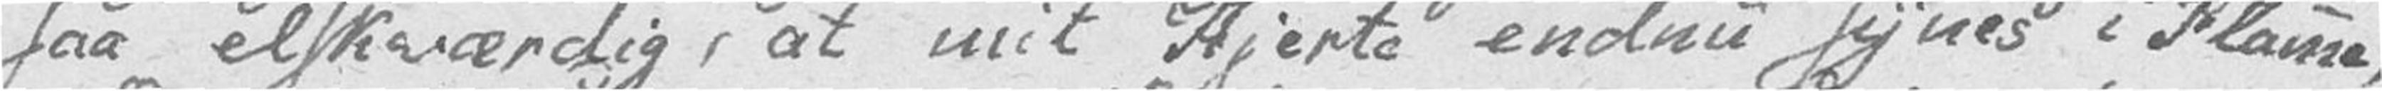saa elskværdig, at mit Hjerte endnu synes i Flamme,
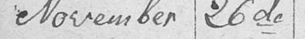November 26de
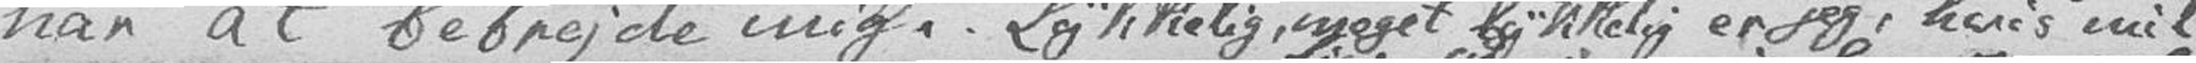har at bebrejde mig. Lykkelig, meget lykkelig er jeg, hvis mit
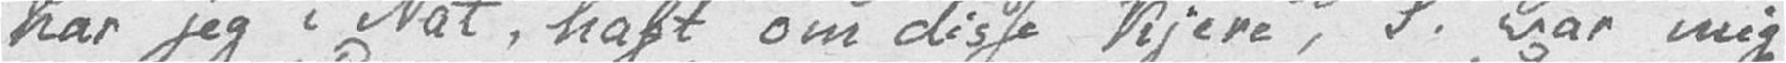har jeg i Nat, haft om disse kjere, S. var mig
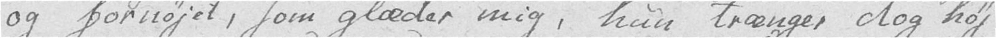og fornøjet, som glæder mig, hun trænger dog høj
